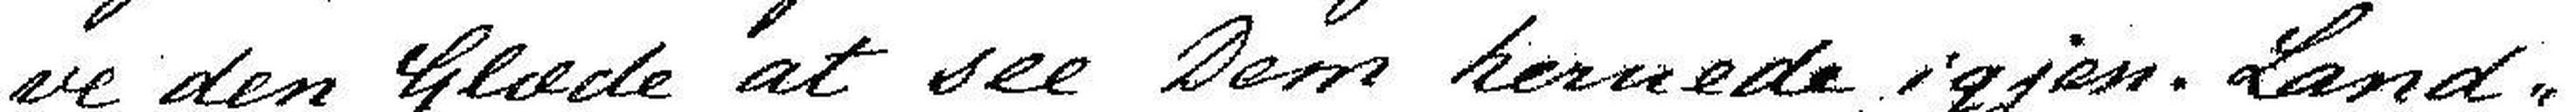ve den Glæde at see Dem hernede igjen. Land-
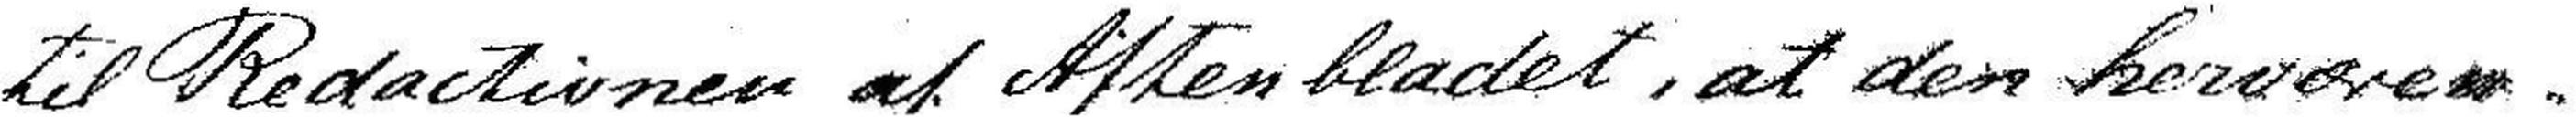til Redactionen af Aftenbladet, at den herværen-
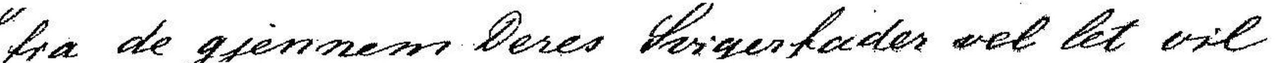fra de gjennem Deres Svigerfader vel let vil
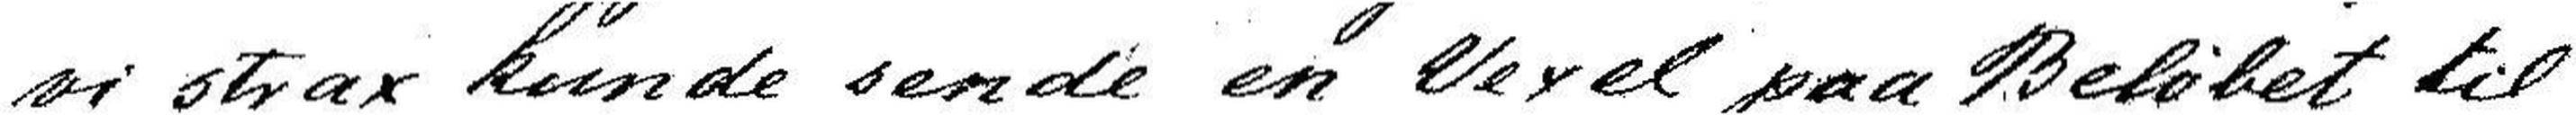vi strax kunde sende en Vexel paa Beløbet til
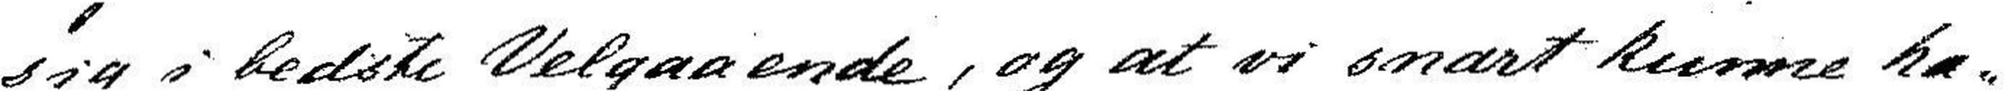sig i bedste Velgaaende, og at vi snart kunne ha-
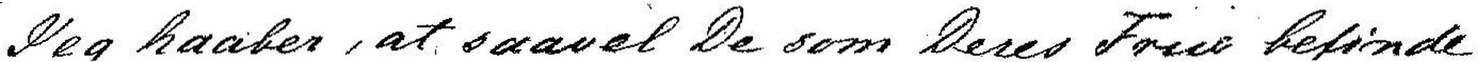Jeg haaber, at saavel De som Deres Frue befinde
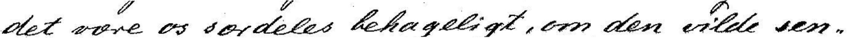det være os særdeles behageligt, om den vilde sen-
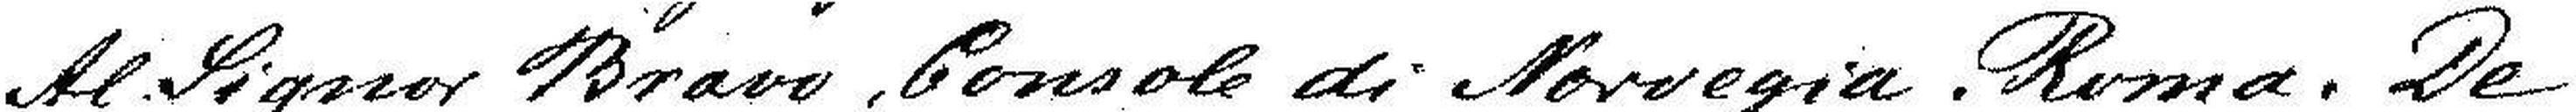Al. Signor Bravo, Console di Norvegia. Roma. De
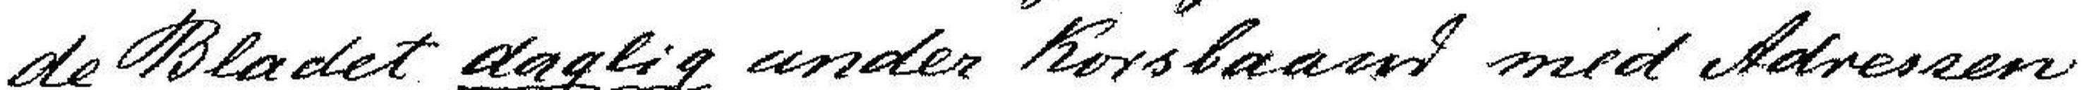de Bladet daglig under Korsbaand med Adressen
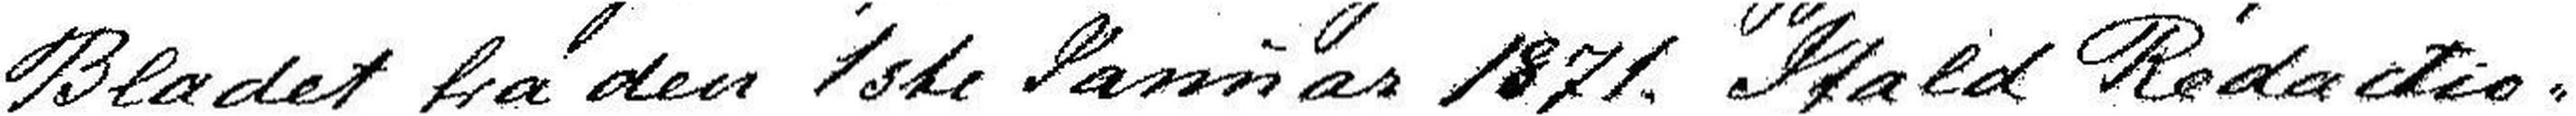Bladet fra den 1ste Januar 1871. Ifald Redactio-
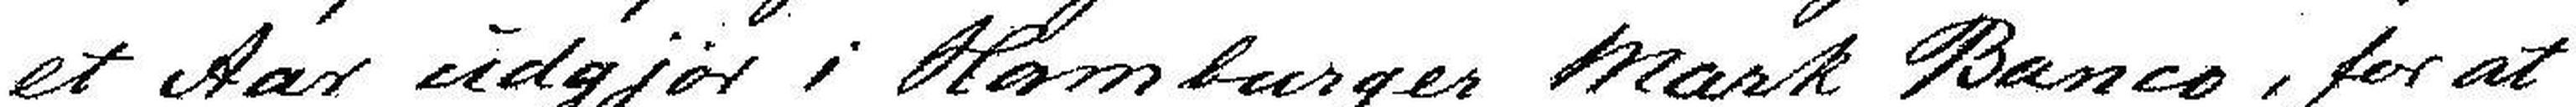et Aar udgjør i Hamburger Mark Banco, for at
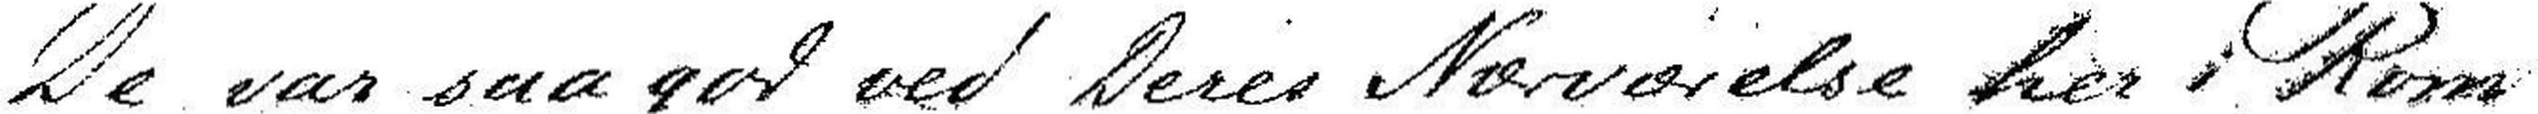De var saa god ved Deres Nærværelse her i Rom
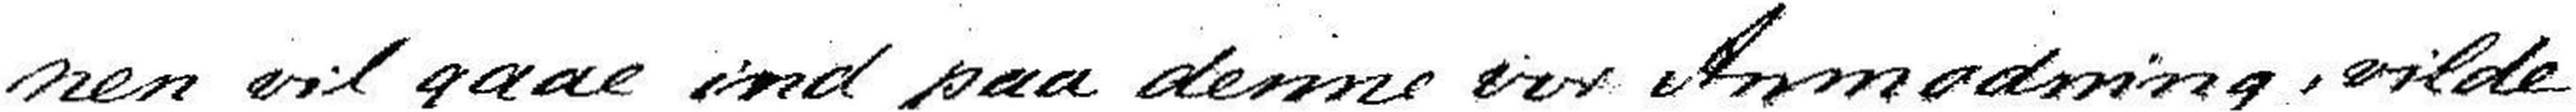nen vil gaae ind paa denne vor Anmodning, vilde
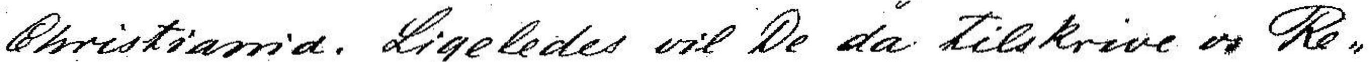Christiania. Ligeledes vil De da tilskrive os Re-
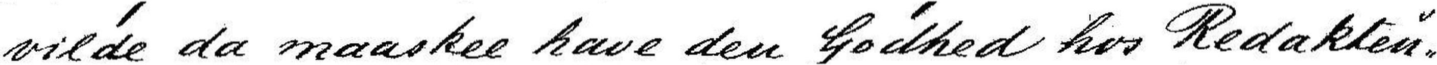vilde da maaske have den Godhed hos Redakteu-
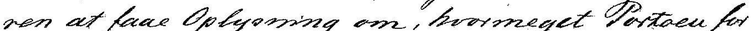ren at faae Oplysning om, hvormeget Portoen for
